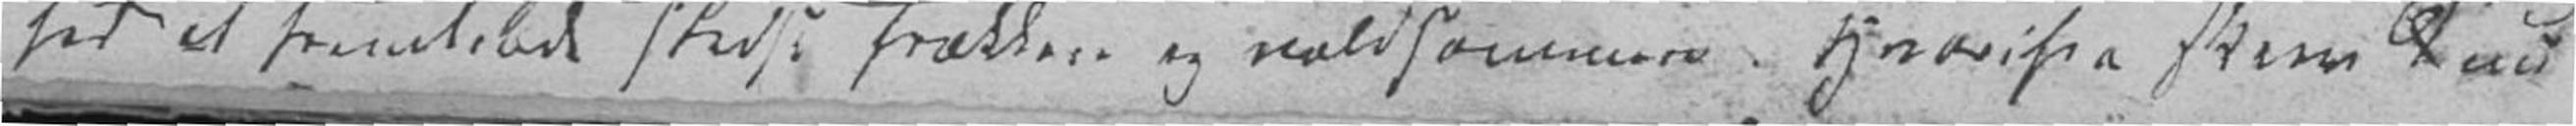hed at fremtræde stedse stærkere og voldsommere. Hvorifra skee d nu
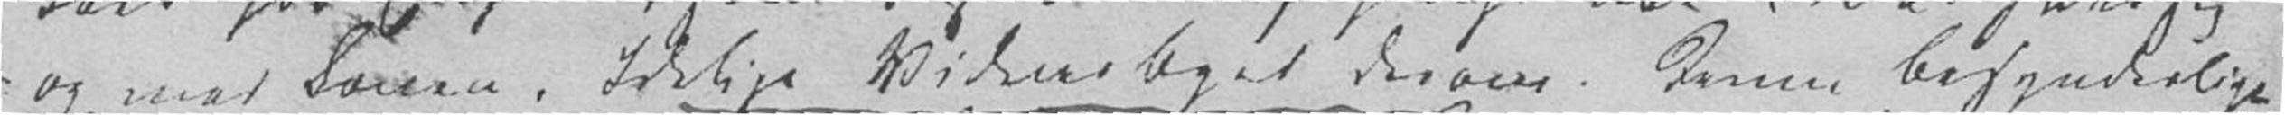op mod Loven. Idelige Vidnesbyrd derom. Denne besynderlige
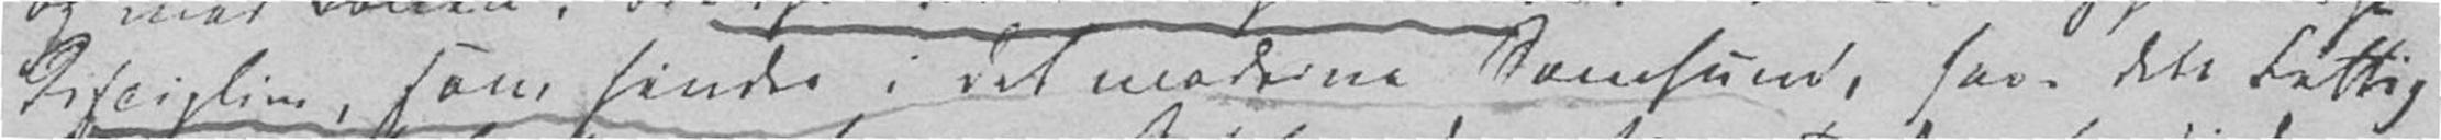Disciplin som findes i det moderne Samfund, hvor dets Fattig-
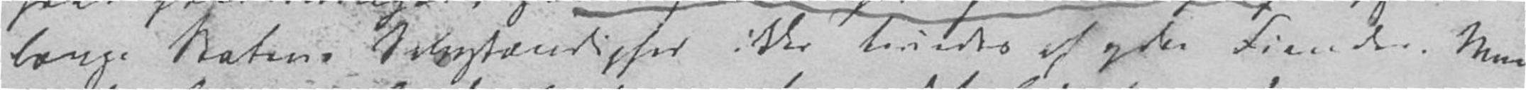længe Statens Selvstændighed ikke truedes af ydre Fiender. Men
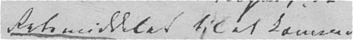Retsmiddelet til at komme
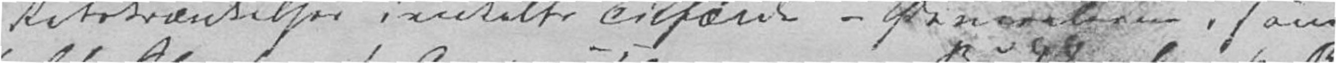Retskrænkelser i enkelte Tilfælde – Semraltum, som
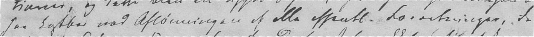saa kostbar ved Afløsningen af alle offentl. Forretninger, de
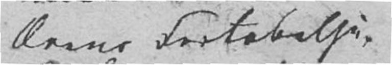Ærens Fortabelse.
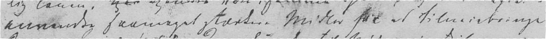anvendte saameget stærkere Midler til at tilveiebringe
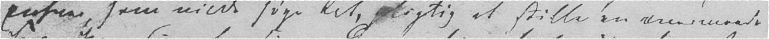enhver som vilde søge Ret, pligtig at stille en overmaade
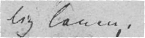lig Loven,
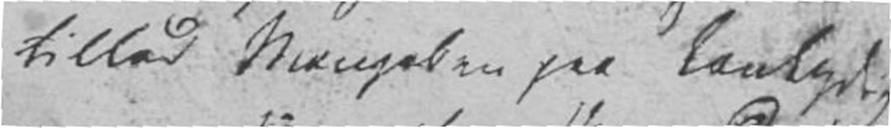tillod Mangelen paa Lovlydig-
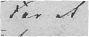For at
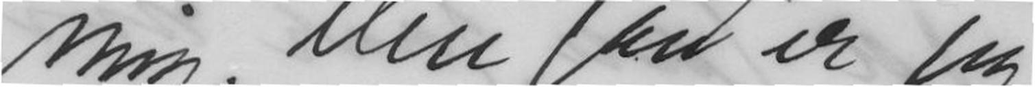mig. Men saa er jeg
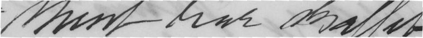=ment har skaffet
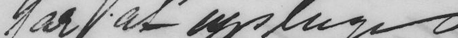for at opsluge
